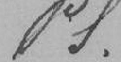PS.
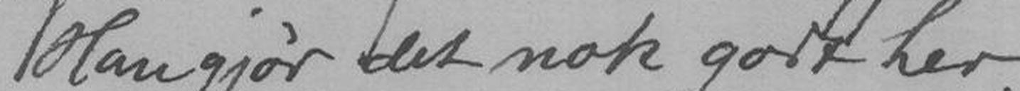Han gjør det nok godt her.
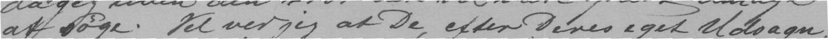at søge. Vel ved jeg at De, efter Deres eget Udsagn,
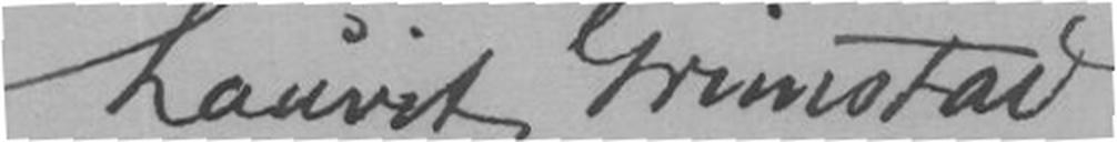Lauritz Grimstad
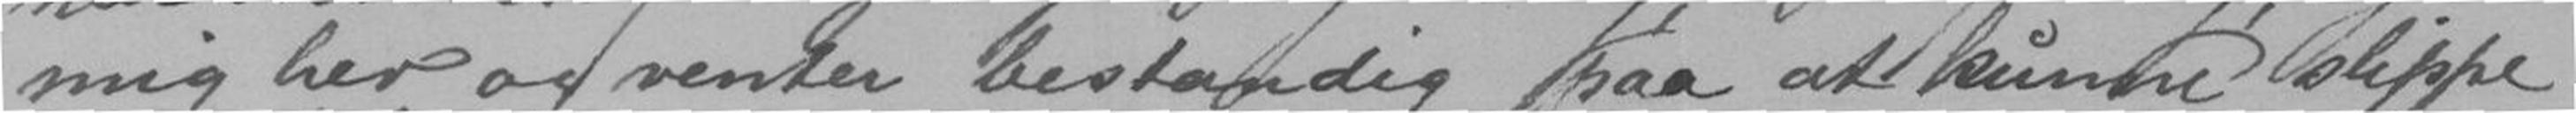mig her og venter bestandig paa at kunde slippe
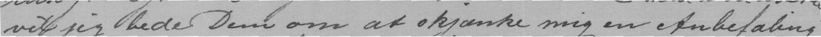vil jeg bede Dem om at skjænke mig en Anbefaling,
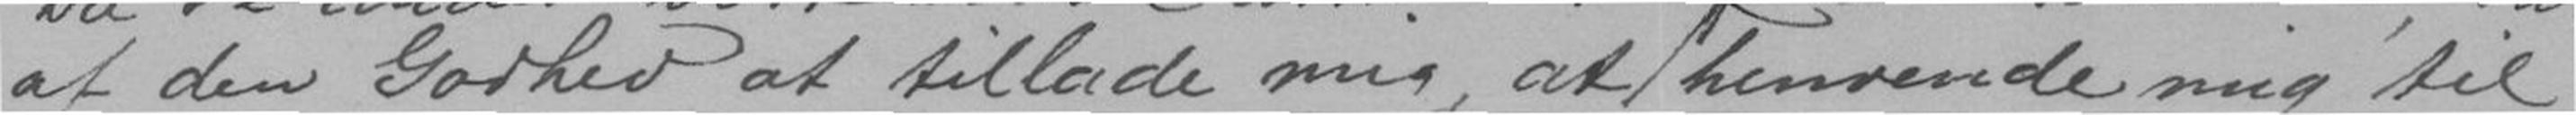af den Godhed at tillade mig at henvende mig til
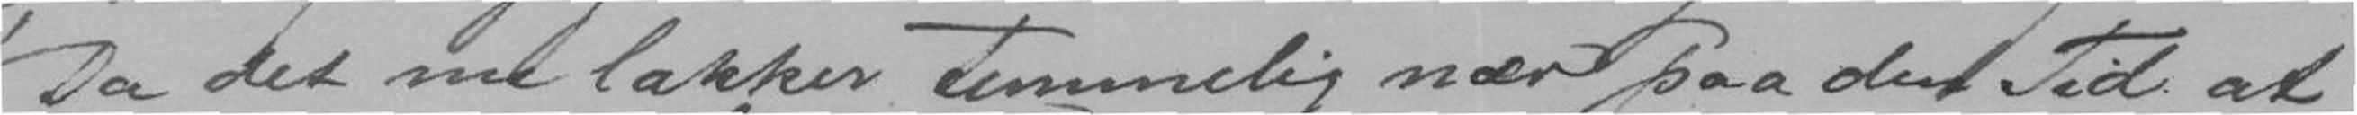Da det nu lakker temmelig nær paa den Tid, at
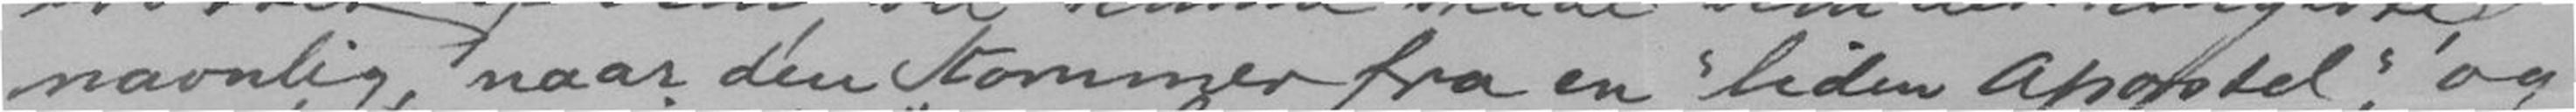navnlig, naar den Kommer fra en "liden apostel", og
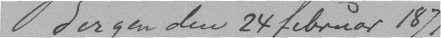Bergen den 24 februar 1874.
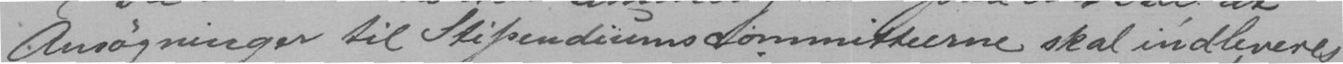Ansøgninger til Stipendiumscommitteerne skal indleveres,
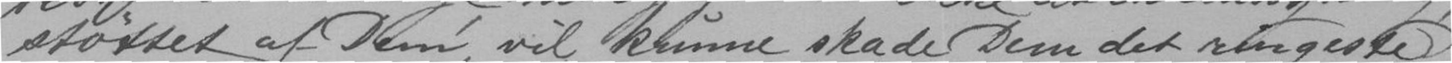støttet af Dem vil kunne skade Dem det ringeste,
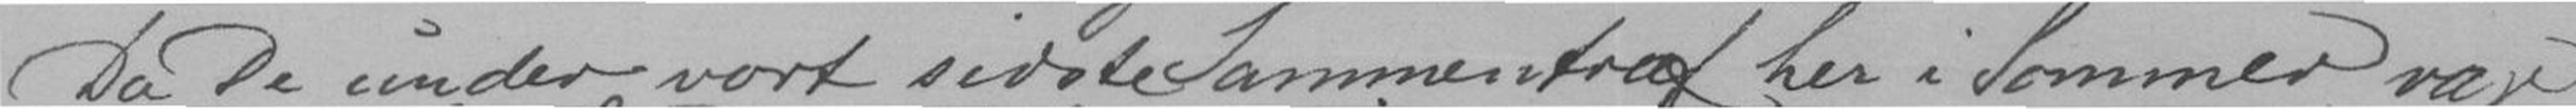Da De under vort sidste Sammentræf her i Sommer var
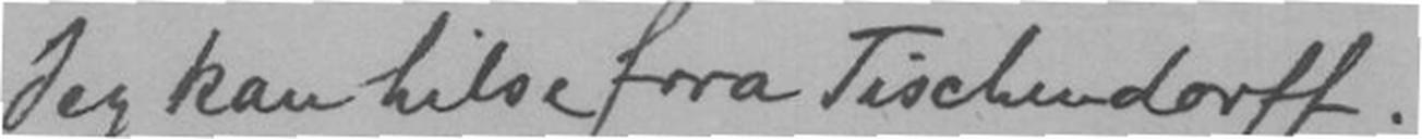Jeg kan hilse fra Tischendorff.
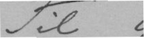Til
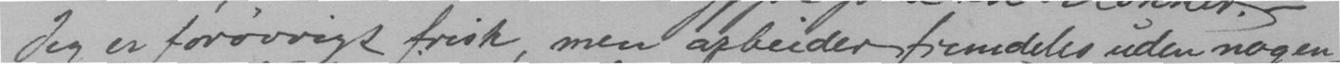Jeg er forøvrigt frisk, men arbeider fremdeles uden nogen-
